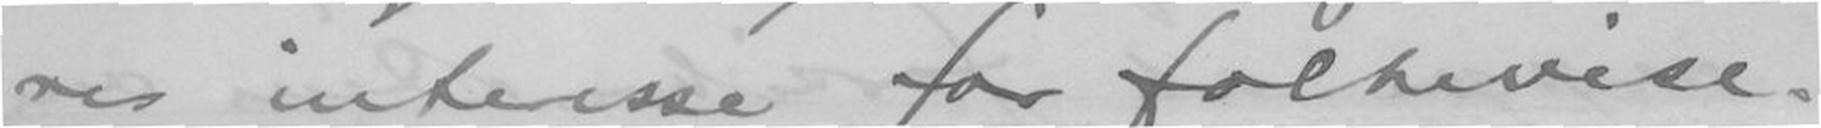res interesse for folkevise-
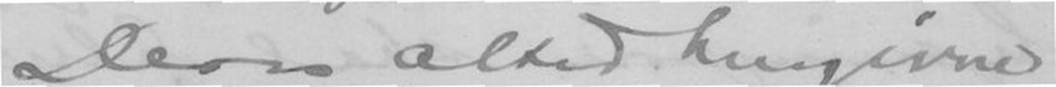Deres altid hengivne
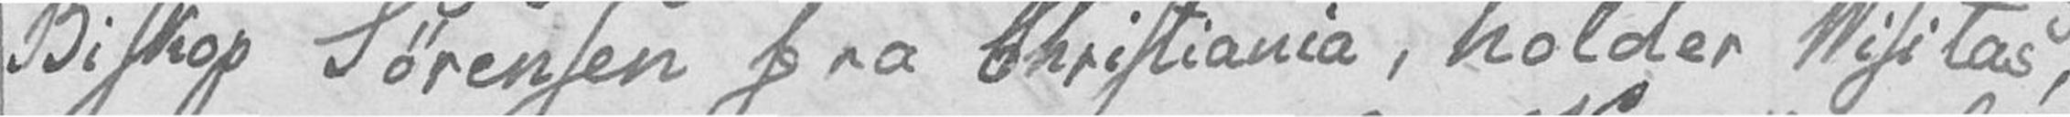Biskop Sørensen fra Christiania, holder Visitas,
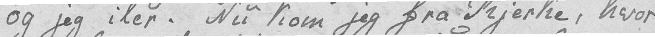og jeg iler. Nu kom jeg fra Kjerke, hvor
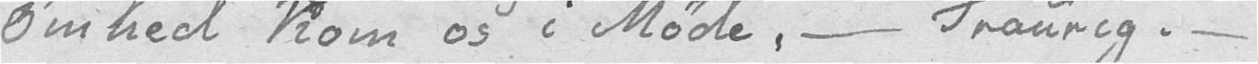Ømhed kom os i Møde. – Traurig. –
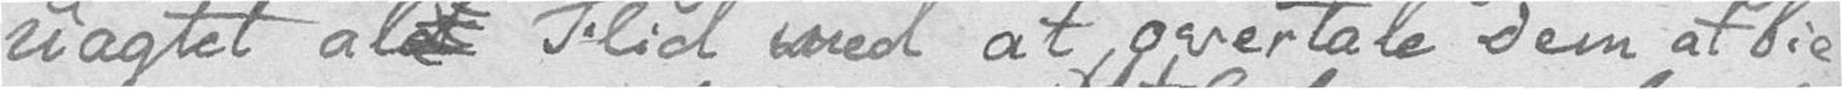uagtet alt Flid med at overtale Dem at bie
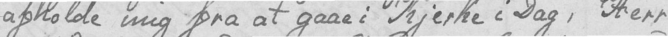afholde mig fra at gaae i Kjerke i Dag, Herr
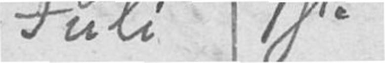Juli 1ste
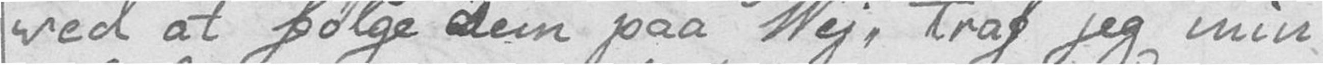ved at følge Dem paa Vej, traf jeg min
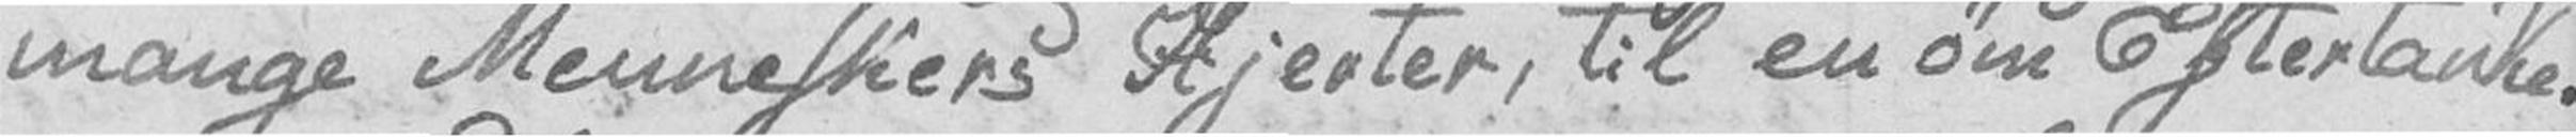mange Menneskers Hjerter, til en øm Eftertanke.
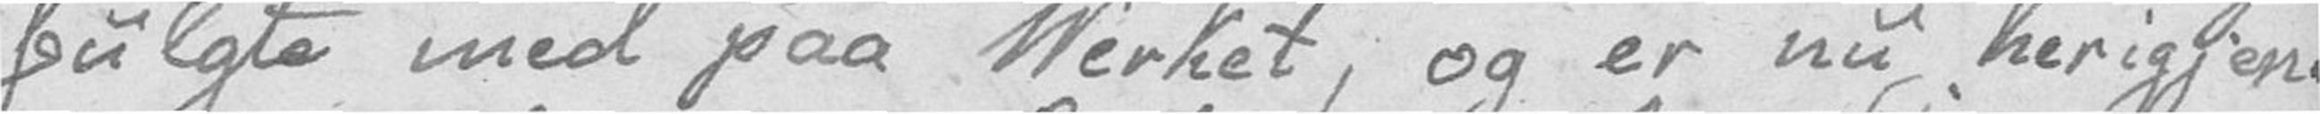fulgte med paa Verket, og er nu her igjen.
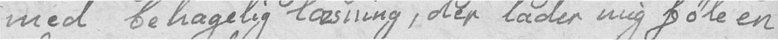med behagelig læsning, der lader mig føle en
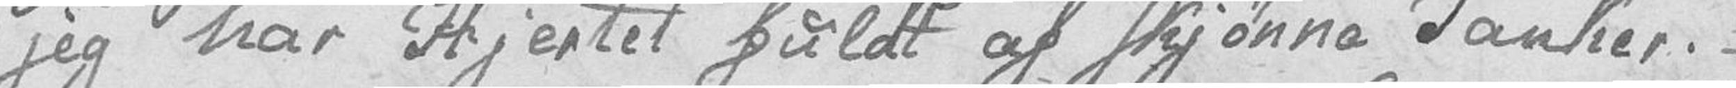jeg har Hjertet fuldt af skjønne Tanker. –
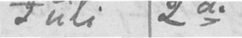Juli 2de
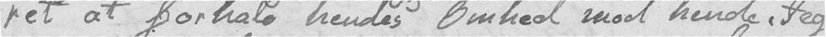ret at forhale hendes Ømhed mod hende. Jeg
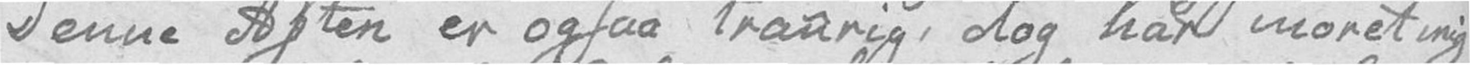Denne Aften er ogsaa traurig, dog har jeg moret mig
